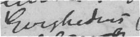Evighedens
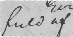fuld af
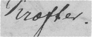Kræfter.
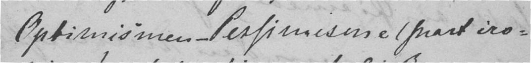Optimismen – Pessimisine (snart iro-
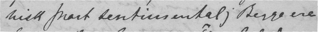isk snart sentimental) Begge ere
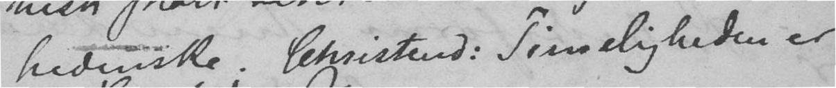hedenske. Christend: Timeligheden er
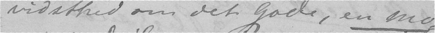vidsthed om det Gode, en mo-
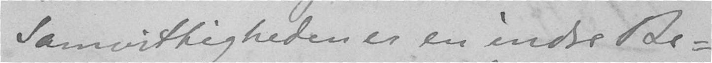Samvittigheden er en indre Be-
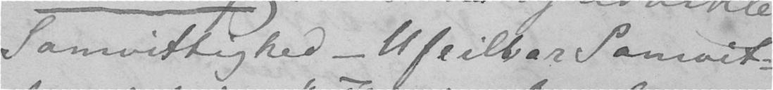Samvittighed - Ufeilbar Samvit-
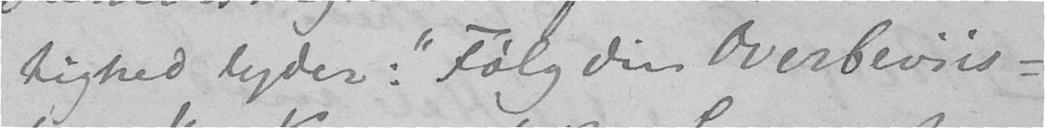tighed lyder: "Følg din Overbeviis-
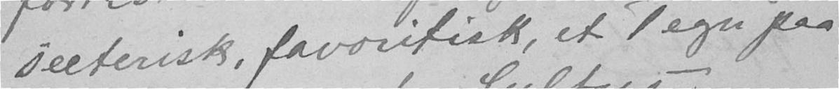secterisk, favoritisk, et Tegn paa
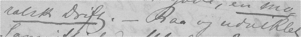ralsk Drift. – Raa og udviklet
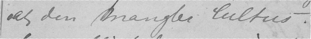at den mangler Cultur.
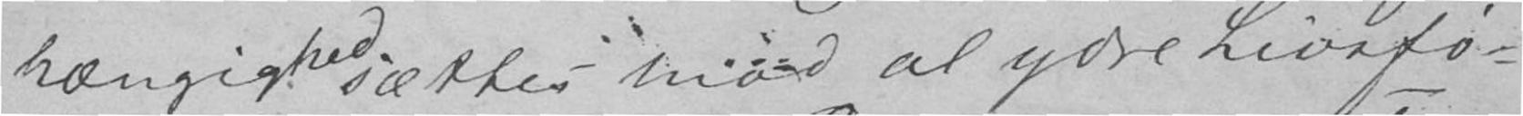hængighed sættes mod al ydre Livsfø-
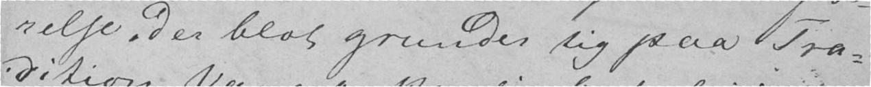relse, der blot grunder sig paa Tra-
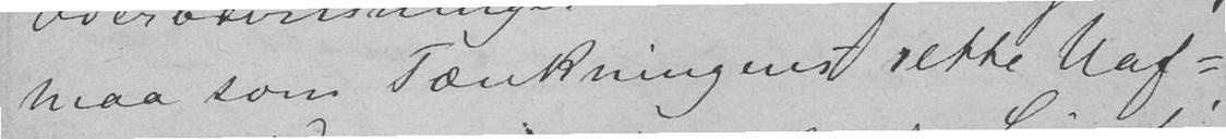maa som Tænkningens rette Uaf-
